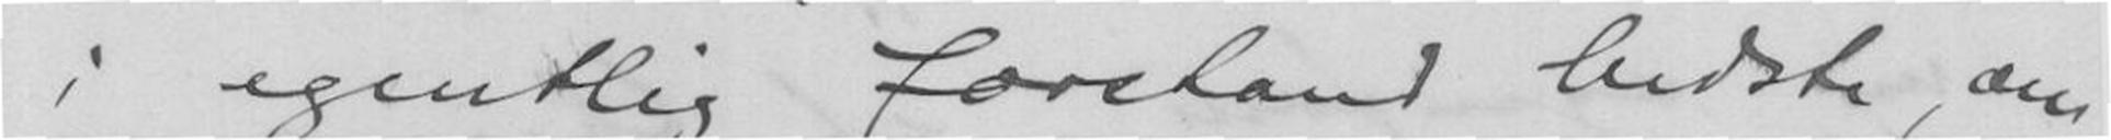i egentlig forstand bedste, om
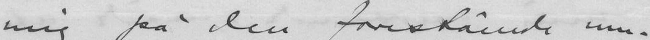mig på den forestående mu-
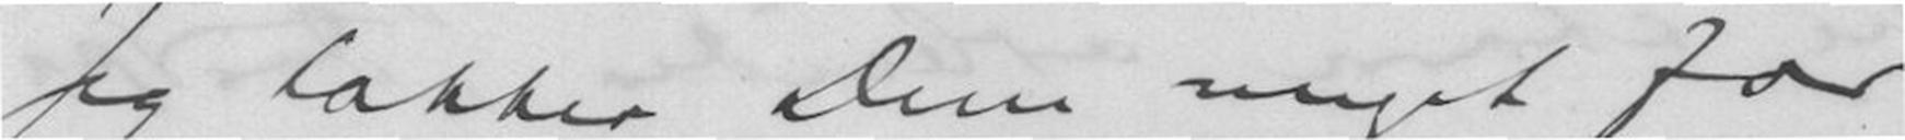Jeg takker Dem meget for
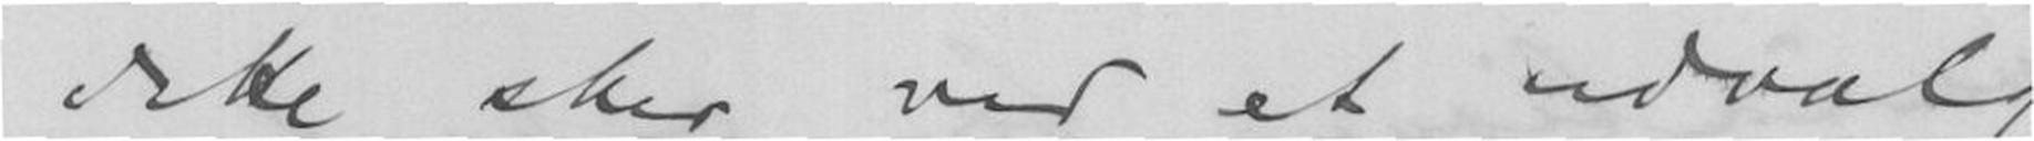dette sker ved et udvalg
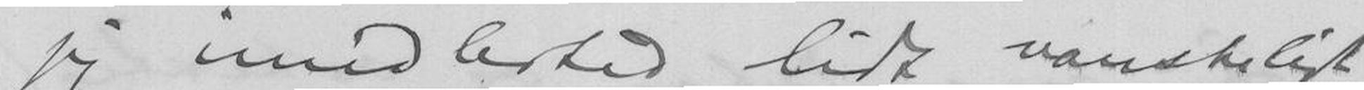jeg imidlertid lidt vanskeligt
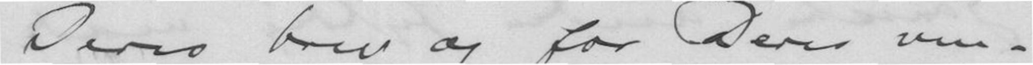Deres brev og for Deres ven-
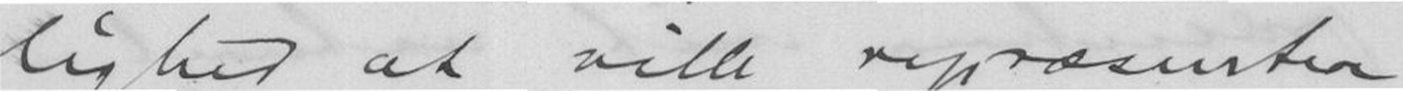lighed at ville repræsentere
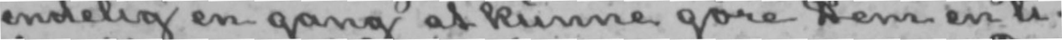endelig en gang at kunne gøre Dem en li-
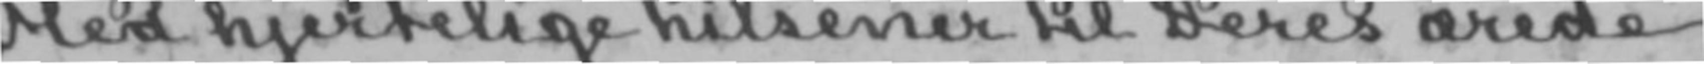Med hjertelige hilsener til Deres ærede
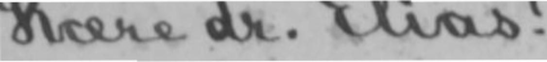Kære dr. Elias!
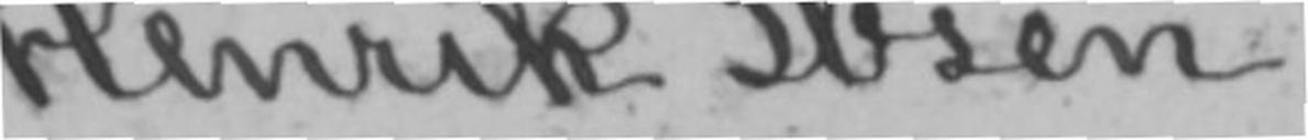Henrik Ibsen
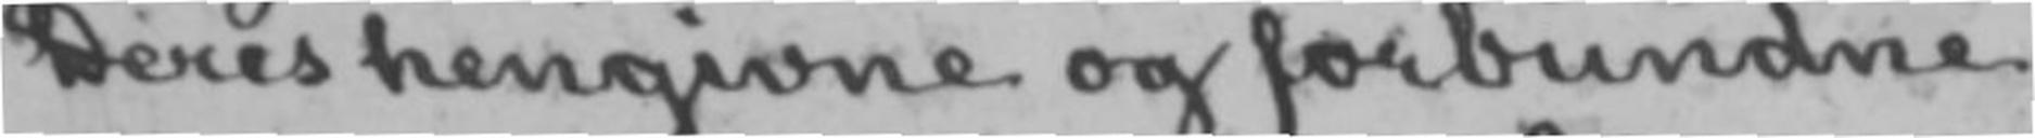Deres hengivne og forbundne
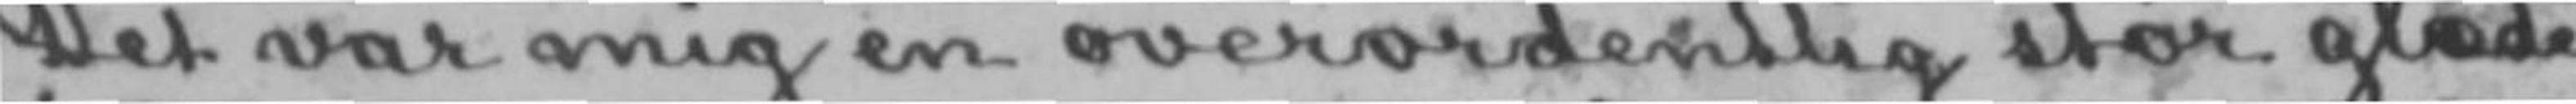Det var mig en overordentlig stor glæde
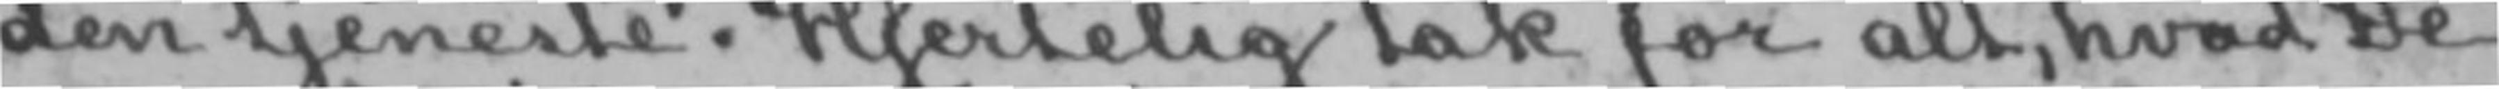den tjeneste. Hjertelig tak for alt, hvad De
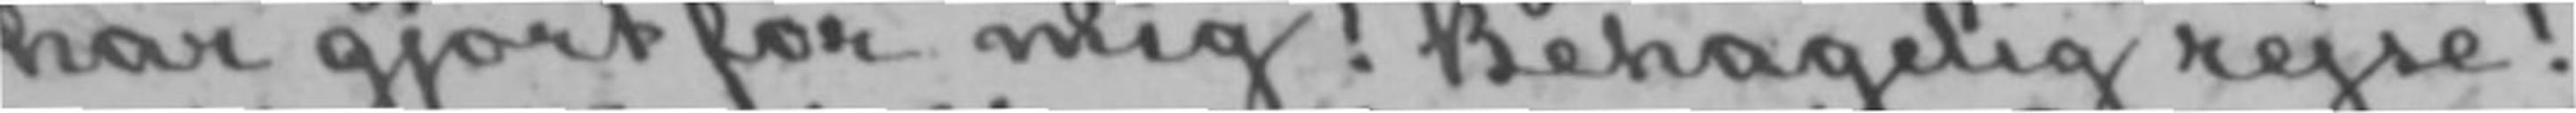har gjort for mig! Behagelig rejse!
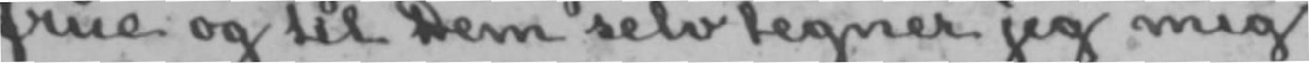frue og til Dem selv tegner jeg mig
